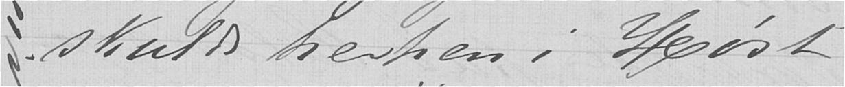skulde herhen i Høst
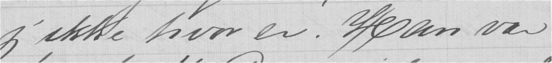jeg ikke hvor er. Han var
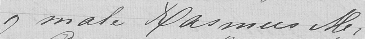og male Rasmus M,
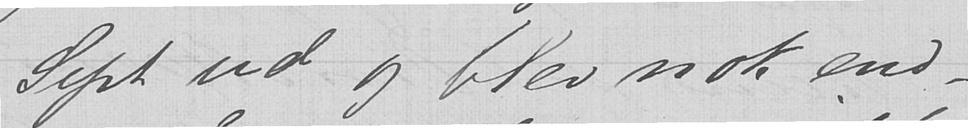Sept ud og blev nok end-
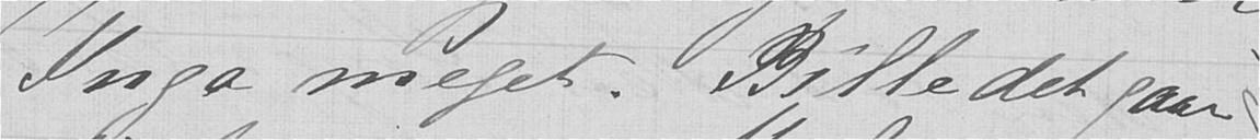Inga meget. Billedet gaar
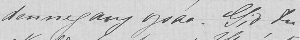dennegang ogsaa. Gid du
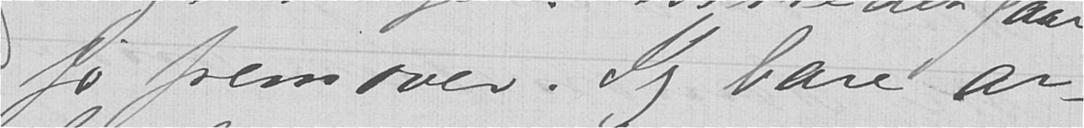jo fremover. Jeg bare ar-
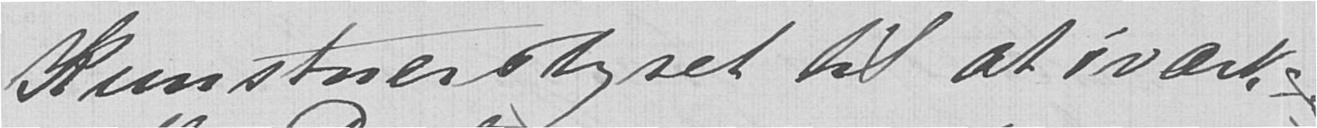Kunstnerstyret til at iværk-
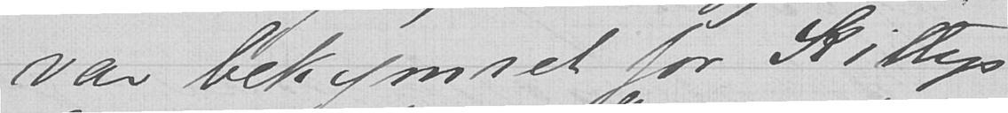var bekymret for Kittys
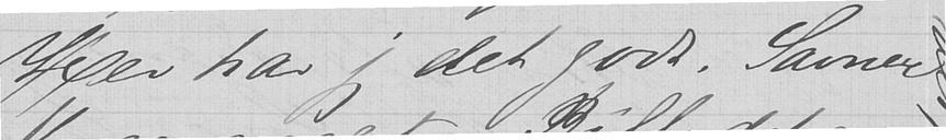Her har jeg det godt. Savner
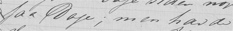faa Dage; men havde
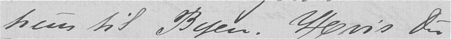hun til Byen. Hvis du
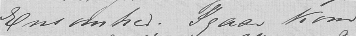Ensomhed. Igaar kom
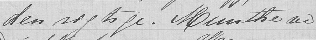den rigtige. Munthe ved
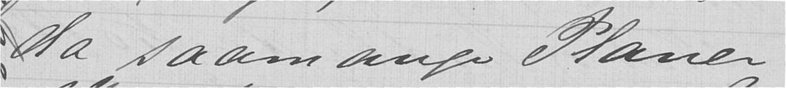da saamange Planer.
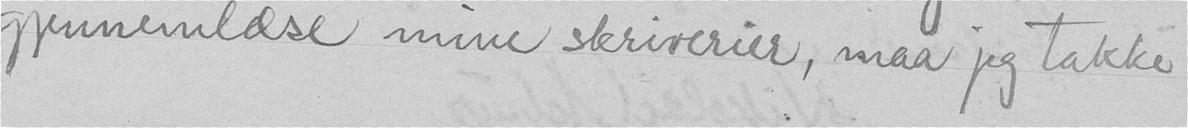gjennnemlæse mine skriverier, maa jeg takke
